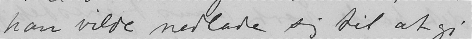han vilde nedlade sig til at gi
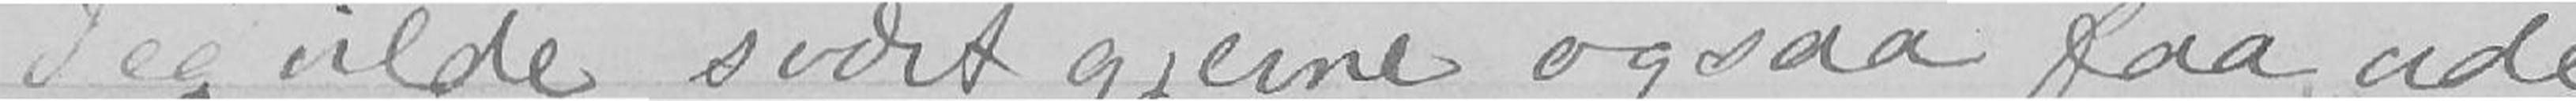Jeg vilde svært gjerne ogsaa faa vide,
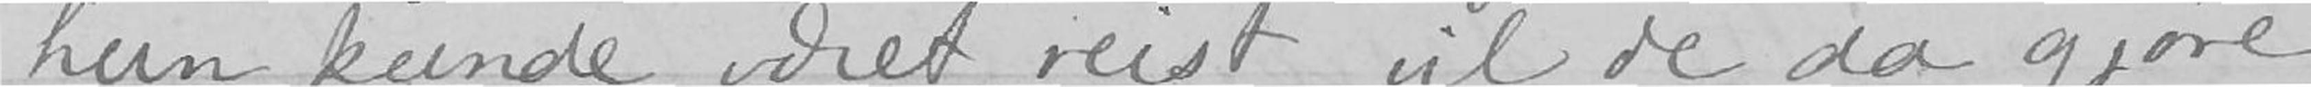hun kunde været reist, vil de da gjøre
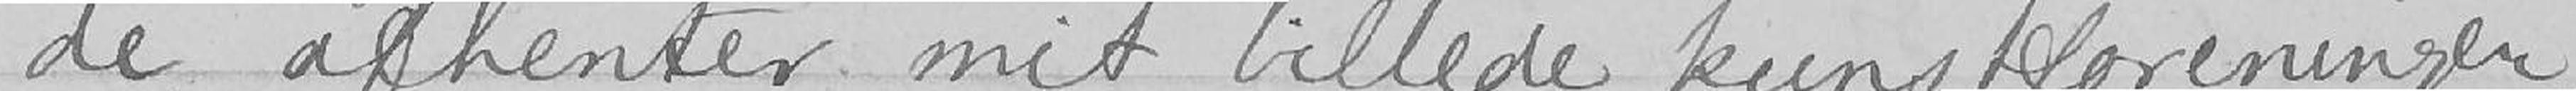de afhenter mit billede kunstforeningen,
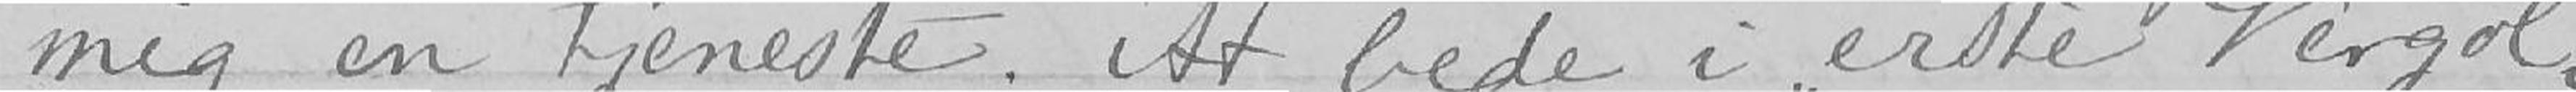mig en tjeneste. At bede i «erste Vergolder-
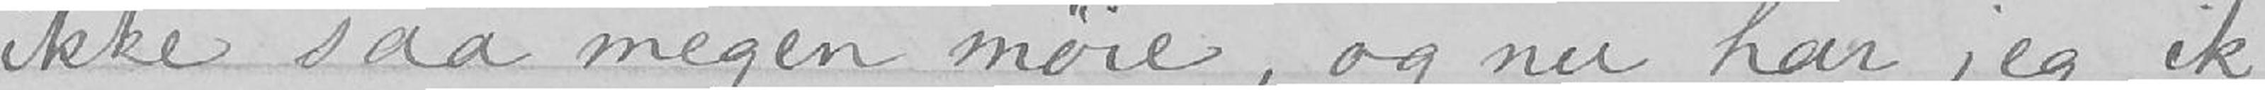ikke saa megen møie, og nu har jeg ik-
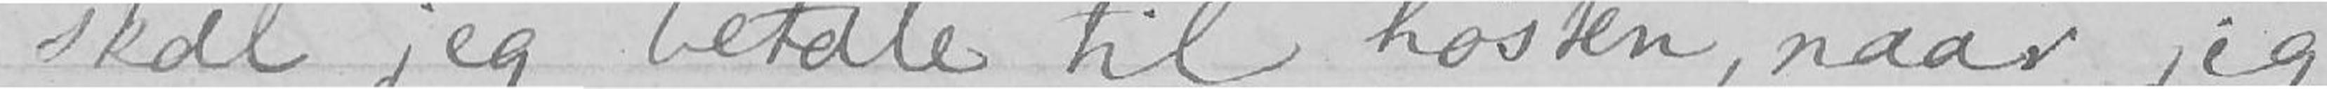skal jeg betale til høsten, naar jeg
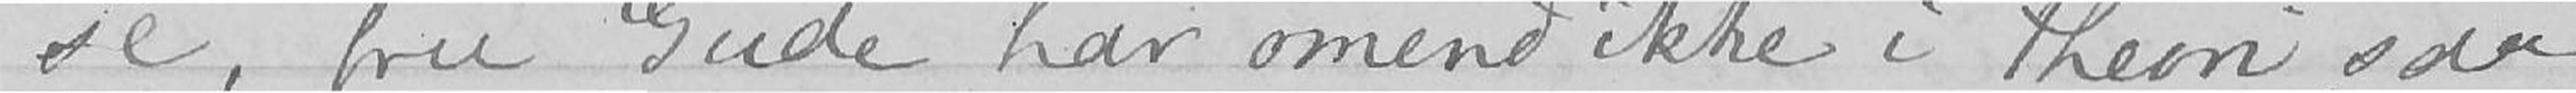se, fru Gude har omend ikke i theori, saa
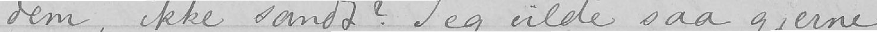dem, ikke sandt? Jeg vilde saa gjerne
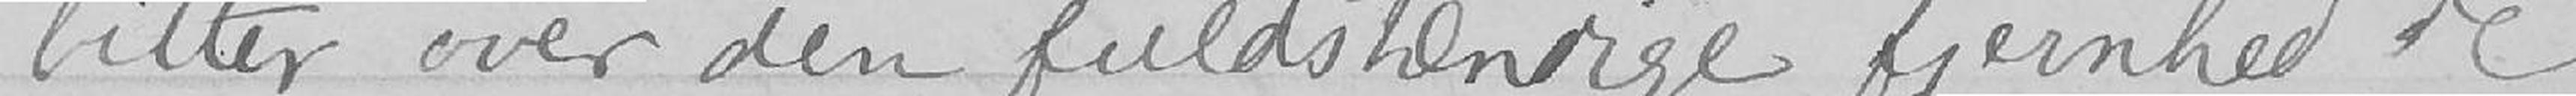bitter over den fuldstændige fjernhed de
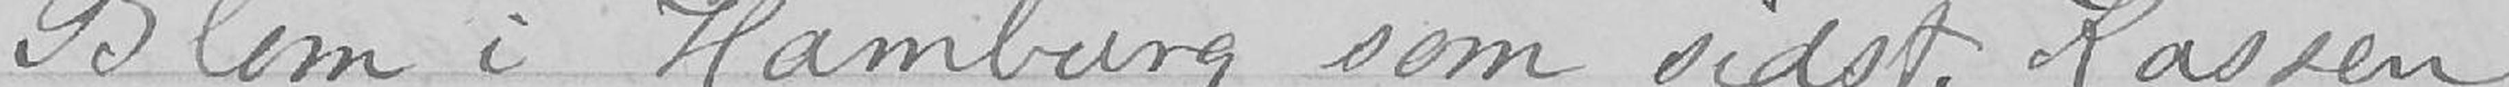Blom i Hamburg som sidst. Kassen
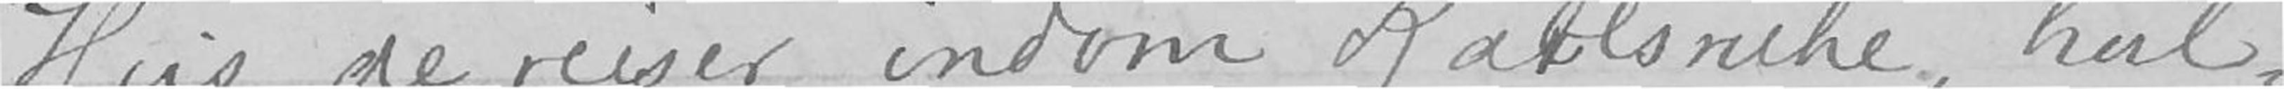Hvis de reiser indom Karlsruhe, hvil-
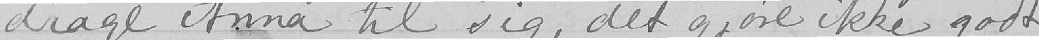drage Anna til sig, det gjøre ikke godt,
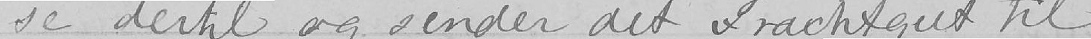se dertil og sender det Frachtgut til
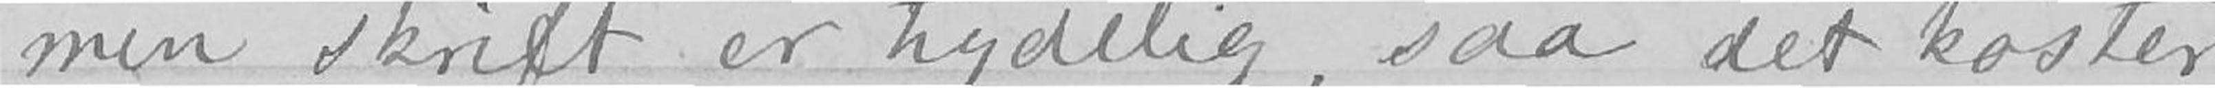min skrift er tydelig, saa det koster
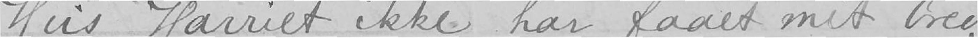Hvis Harriet ikke har faaet mit brev,
